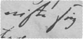viste sig
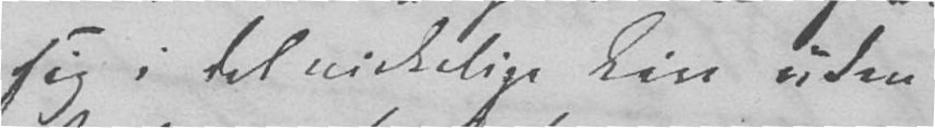sig i det virkelige Liv uden
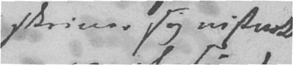skriver sig vistnok
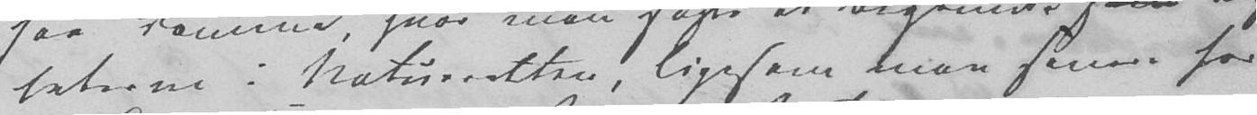taterne i Naturetten, ligesom man seneree har
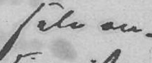selv om-
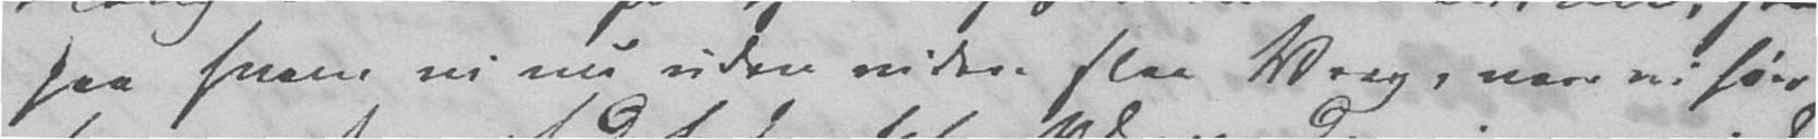paa hvem vi nu uden videre slaa Vrag, naar vi høre
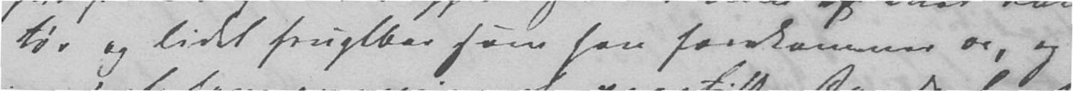tør og lidet frugtbar som han forekommer os, og
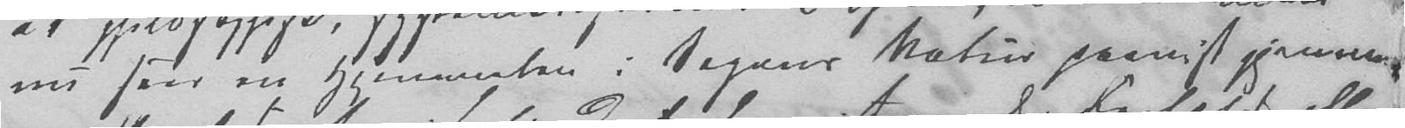nu seer an Hjemmelen i Sagens Natur paavist gjennem
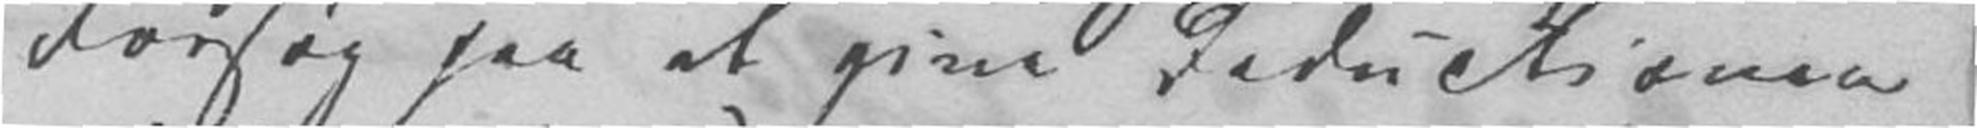Forsøg paa at give Deductioner
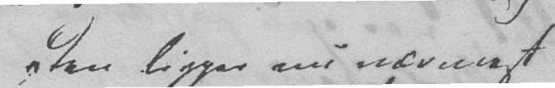Den ligger nu nærmest
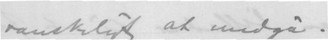vanskeligt at medgå.
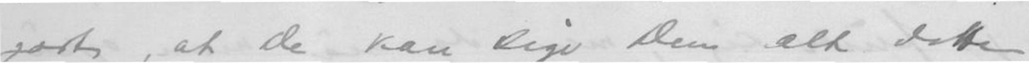godt, at De kan sige Dem alt dette
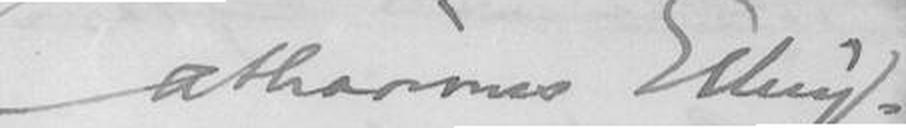Catharinus Elling
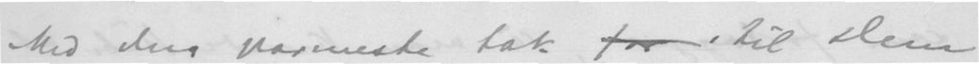Med den varmeste tak for, til Dem
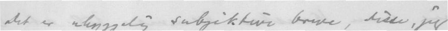Det er uhyggelig subjektiv breve, disse, jeg
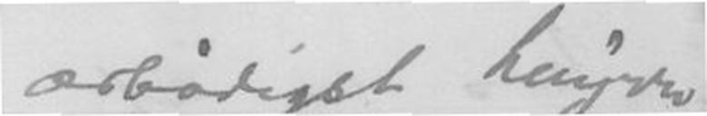ærbødigste hengivne
